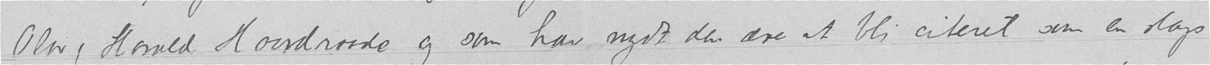Olav og Harald Haardraade og som har nydt den ære at bli citeret som en slags
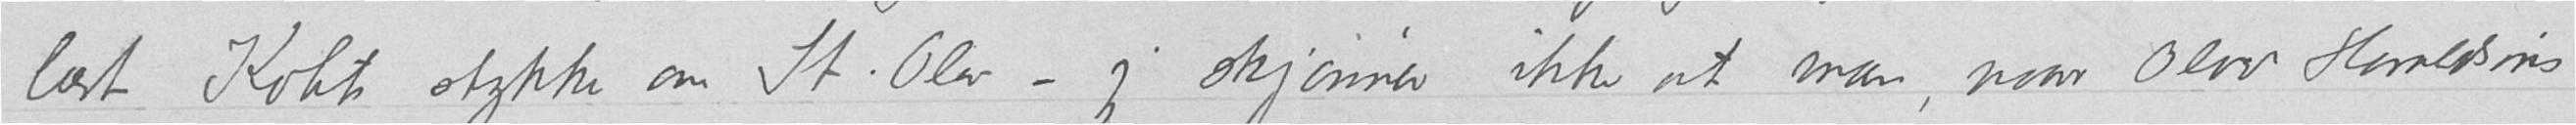læst Kohts stykke om St. Olav – jeg skjønner ikke at man, naar Olav Haraldsøns
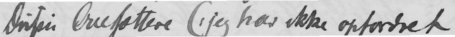Dusin Oversættere (jeg har ikke opfordret
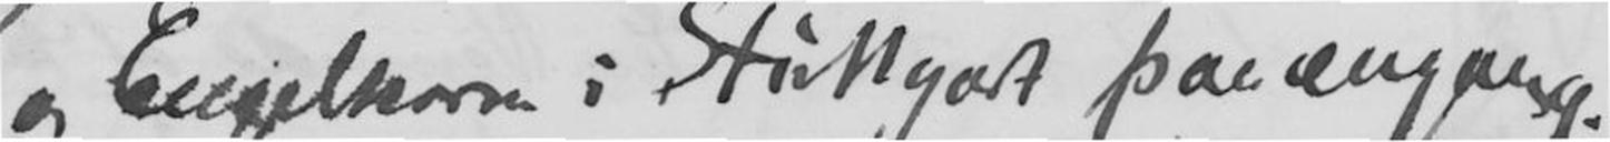og Engelhorn i Stüttgart paa engang.
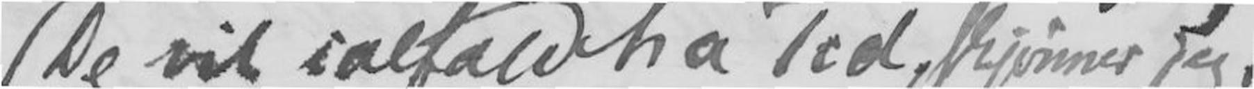De vil ialfald ha Tid, skjønner jeg.
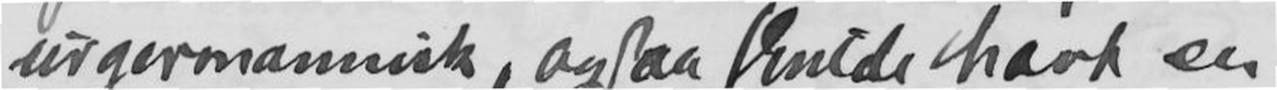urgermannisk, ogsaa skulde havt en
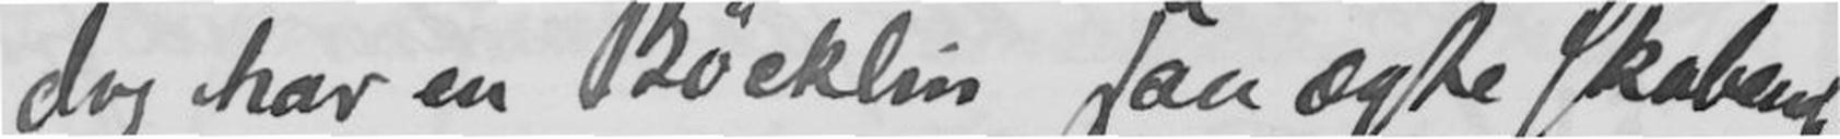dog har en Bøcklin saa ægte skabende
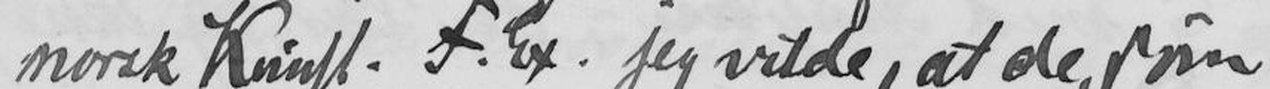norsk Kunst. F. ex. jeg vilde, at de, som
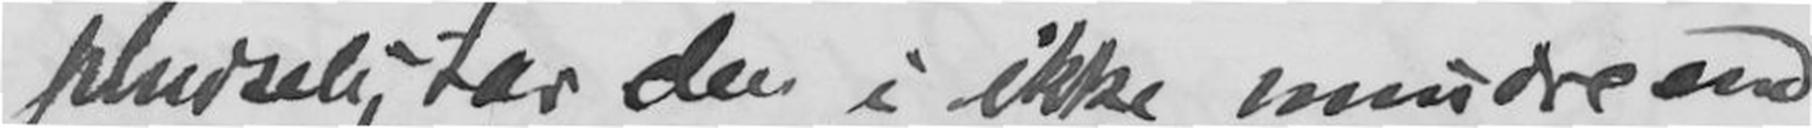pludselig tar den i ikke mindre end
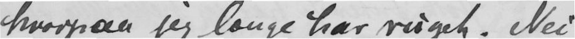hvorpaa jeg længe har ruget. Nei,
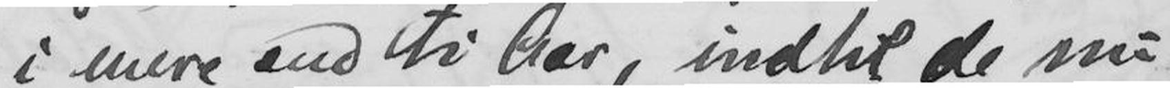i mere end ti Aar, indtil de nu
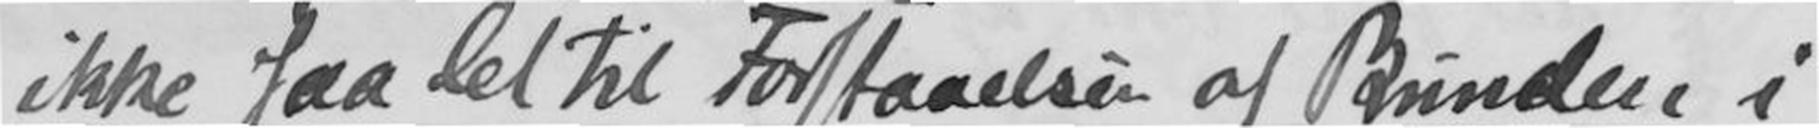ikke saa let til Forstaaelsen af Bunden i
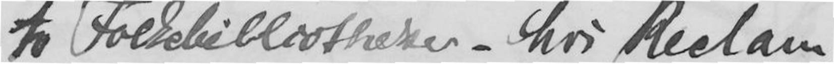to Folkebibliotheker - hos Reclam
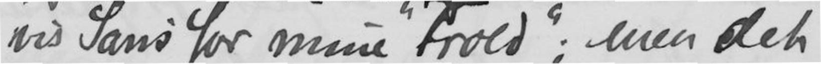vis Sans for mine "Trold"; men det
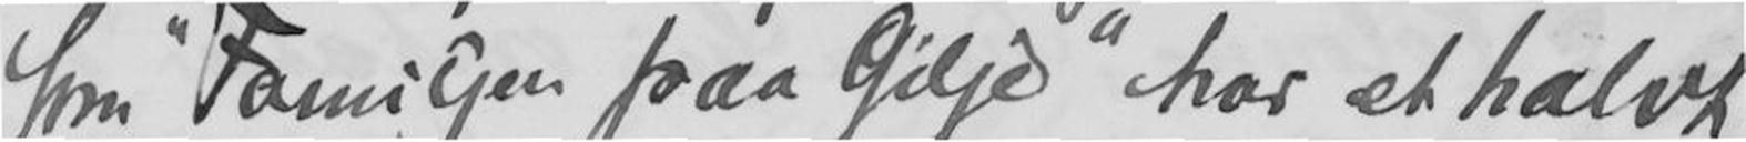som "Familjen paa Gilje" har et halvt
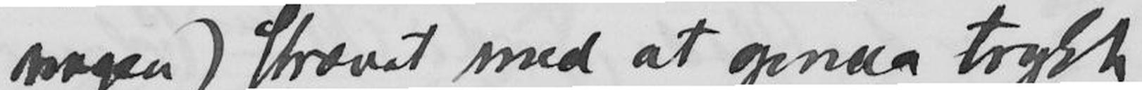nogen) strævet med at opnaa trykt
